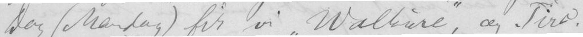Dag (Mandag) fik vi Walkure, og Tirs-
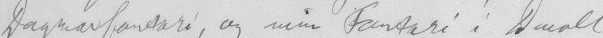Dagmarfantasi, og min Fantasi i Dmoll;
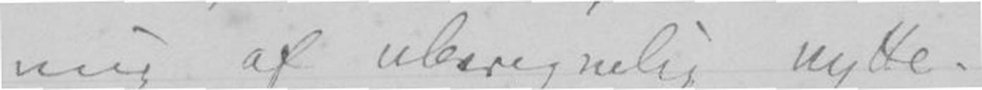mig af uberegnelig nytte.
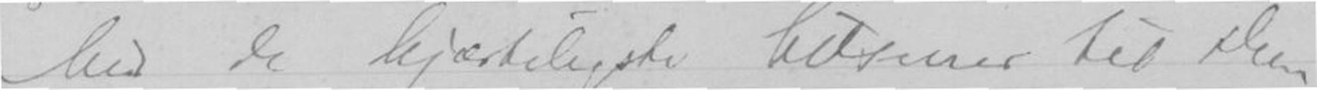Med de hjærteligste hilsener til Dem
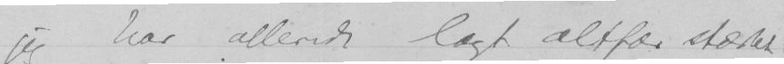jeg har allerede lagt altfor stærkt
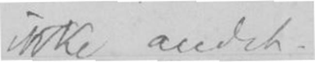ikke andet.
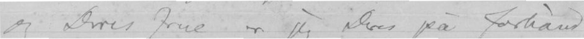og deres frue er jeg Deres på forhånd
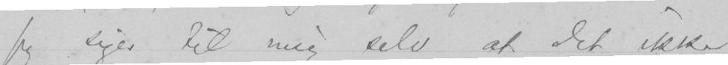Jeg siger til mig selv at det ikke
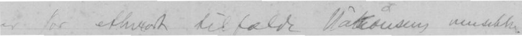er for ethvert tilfælde Håkonsens musikk.
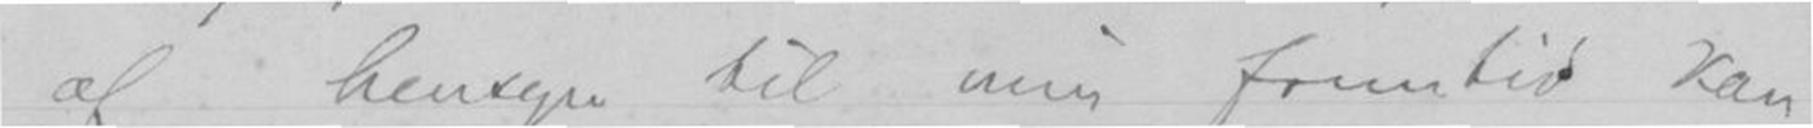af hensyn til min fremtid kan
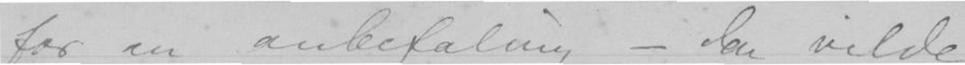for en anbefaling - han vilde
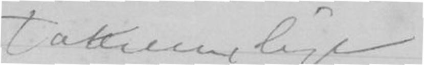taknemlige
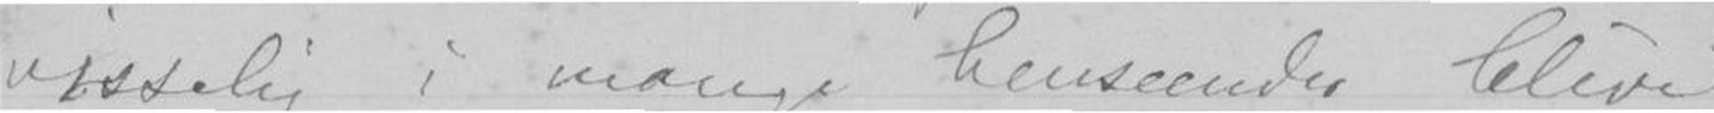visselig i mange henseende blive
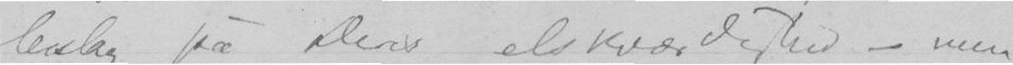beslag på Deres elskværdighed - men
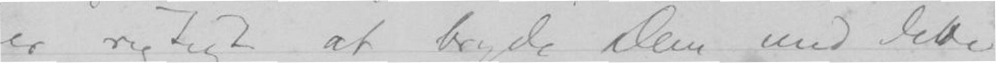er rigtigt at bryde Dem med dette
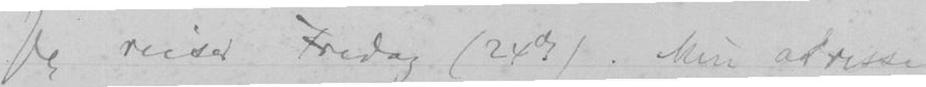Jeg reiser Fredag (24de). Min adresse
